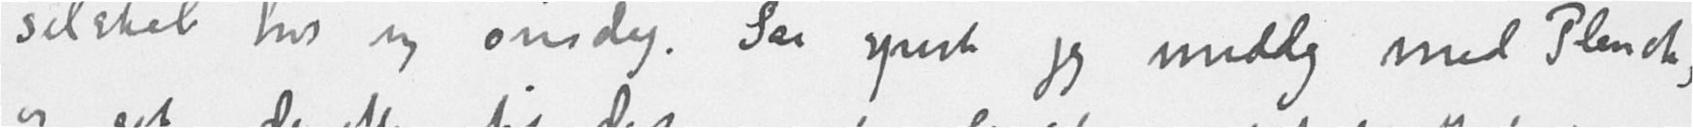selskab hos sig onsdag. Saa spiste jeg middag med Planck,
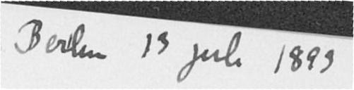Berlin 13 juli 1893
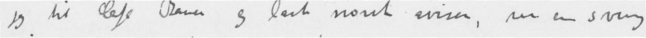jeg til Cafe Barer og læste norske aviser, saa en sving
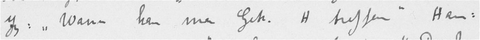Jeg: "Wann kan maa Geh. H treffen" Han:
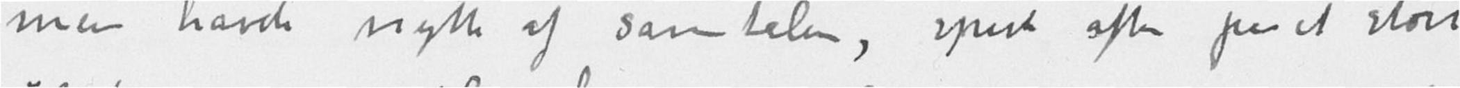men havde nytte af samtalen, spiste aften paa et stort
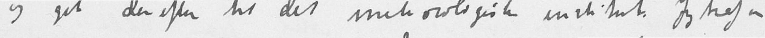og gik derefter til det meteorologiske institut. Jeg traf en
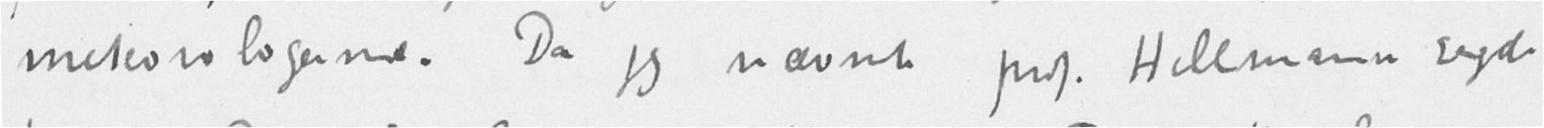meteorologerne. Da jeg nævnte prof. Hillmann sagde
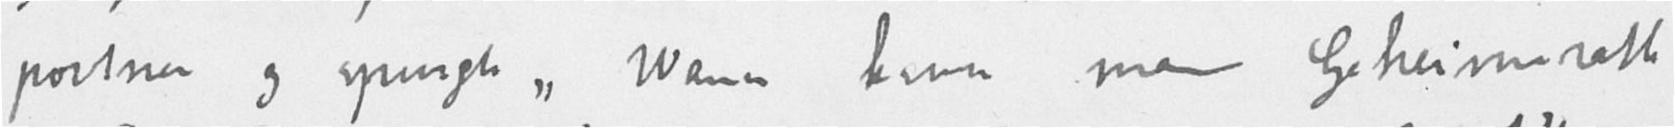portner og spurgte "Wann kann man Geheimerath
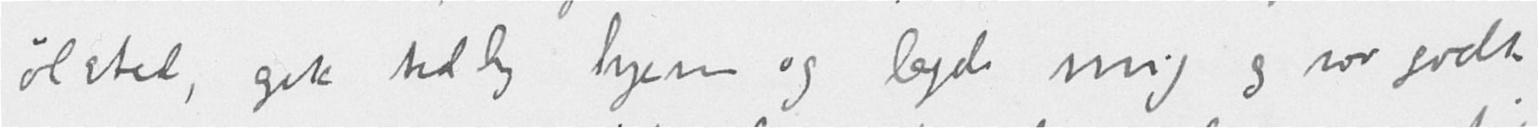ølsted, gik tidlig hjem og lagde mig og sov godt.
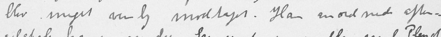blev meget venlig modtaget. Han anordnede aften-
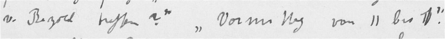v. Bezold treffen?" "Vormittag von 11 bis 1."
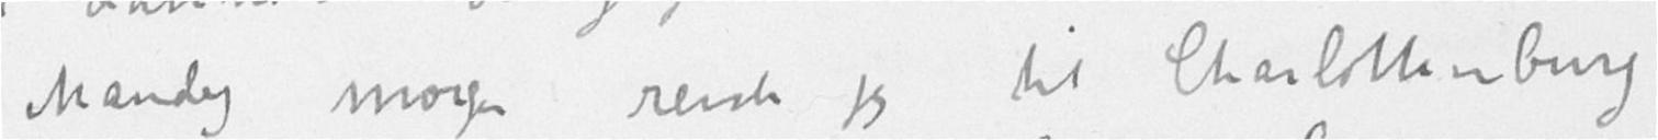Mandag morgen reiste jeg til Charlottenburg
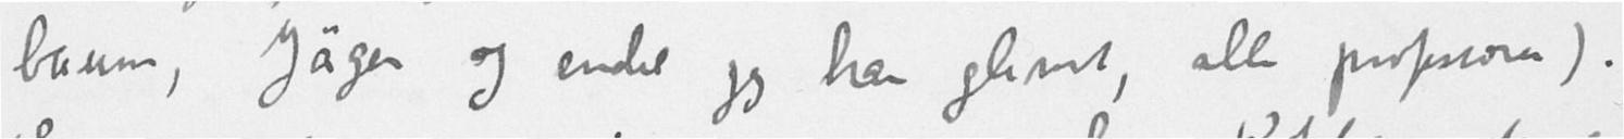baum, Jäger og endel jeg har glemt, alle professorer).
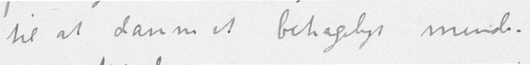til at danne et behageligt minde.
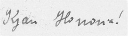Kjære Honoria!
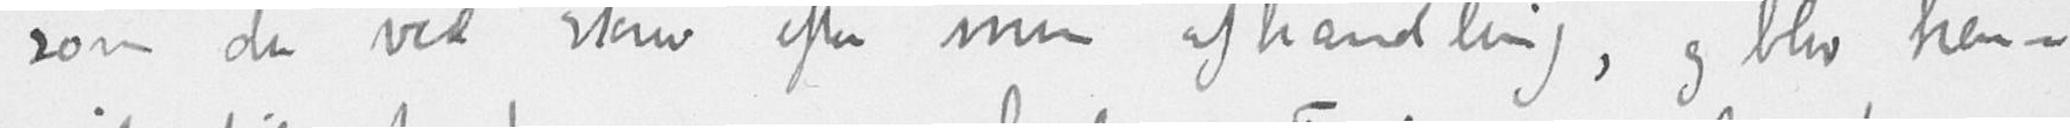som du ved skrev efter min afhandling, og blev hen-
